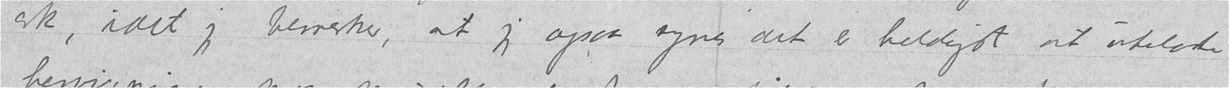ark, idet jeg bemerker, at jeg ogsaa synes det er heldigst at utelade
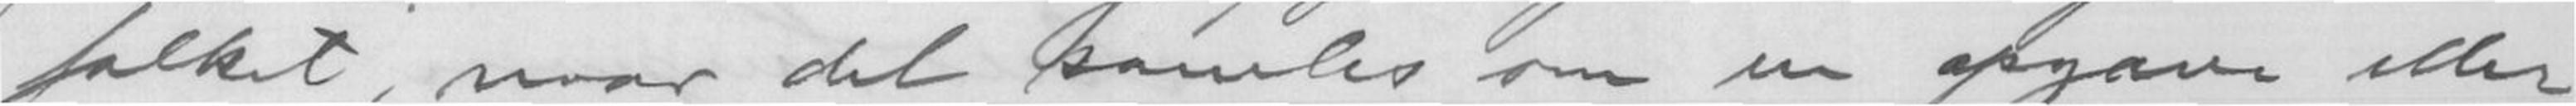folket, naar det samles om en opgave eller
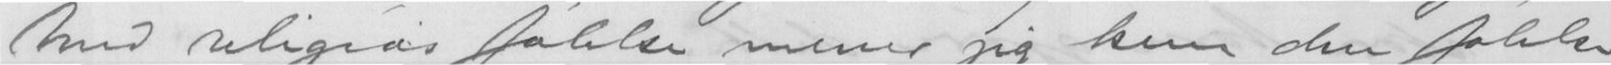Med religiøs følelse mener jeg kun den følelse
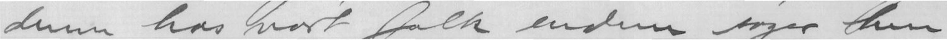denne hos vort folk endnu søger hen
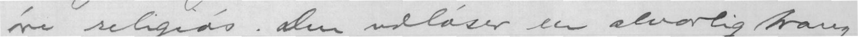øre religiøs. Den udløser en alvorlig trang
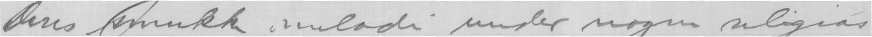Deres smukke melodi under nogen religiøs
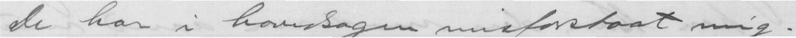De har i hovedsagen misforstaaet mig.
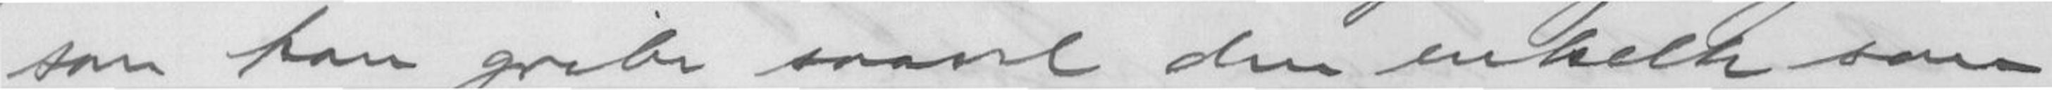som kan gribe saavel den enkelte som
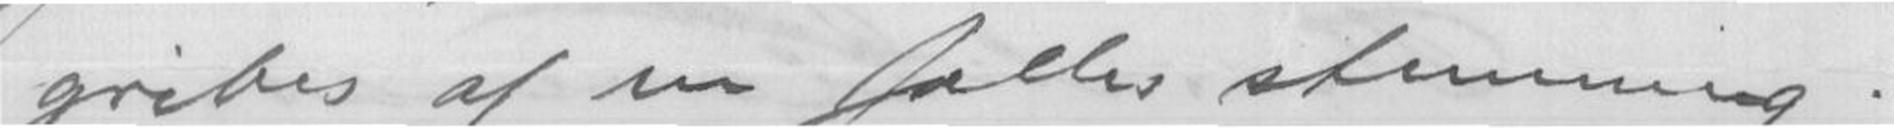gribes af en fælles stemning.
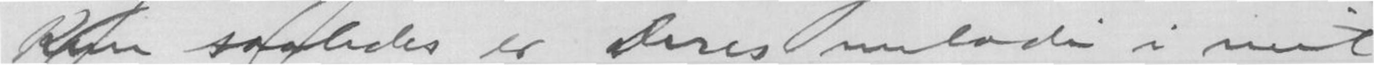Kun saaledes er Deres melodi i mit
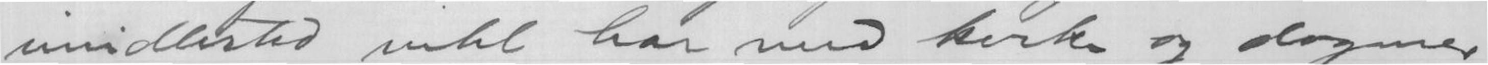imidlertid intet har med kirke og dogmer
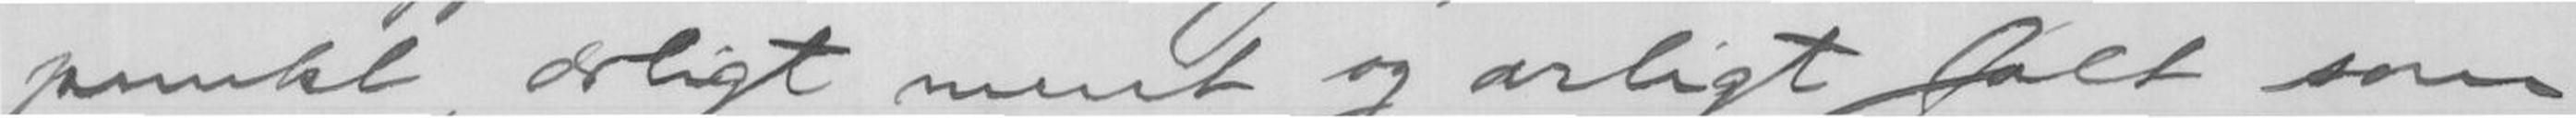punkt, ærligt ment og ærligt talt som
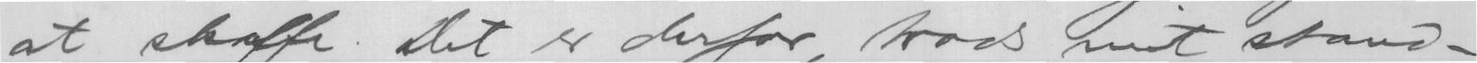at skaffe. Det er derfor, trods mit stand-
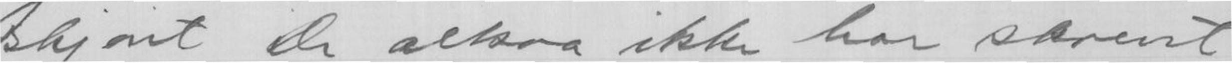skjønt De altsaa ikke har særent
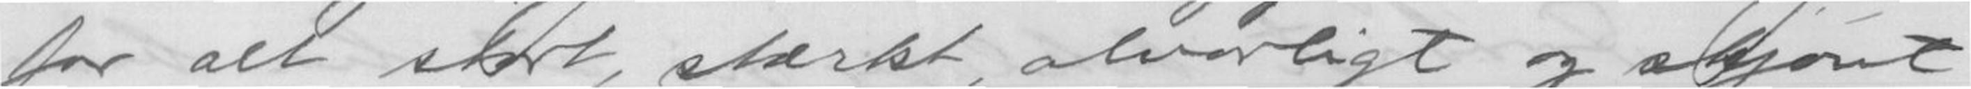for alt stort, stærkt, alvorligt og skjønt
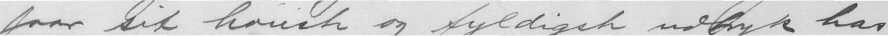faar sit høieste og fyldigste udtryk hos
